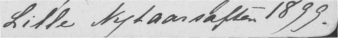Lille Nytaarsaften 1899
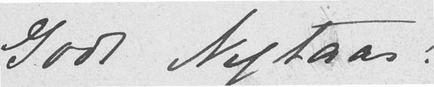Godt Nytaar!
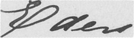Eders
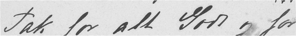Tak for alt Godt og for
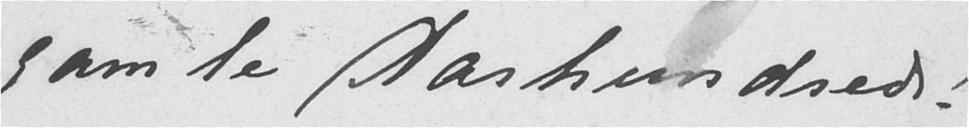gamle Aarhundrede!
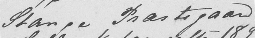Stange Præstegaard
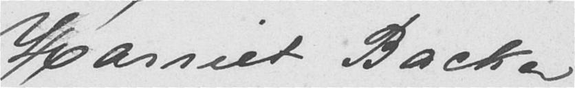Harriet Backer
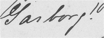Garborg!
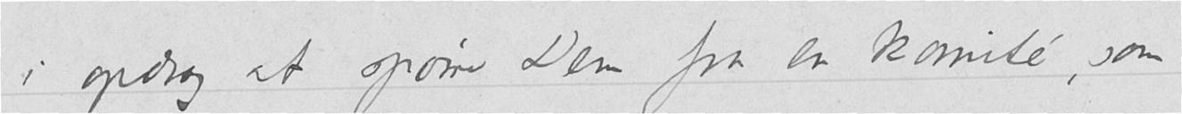i opdrag at spørre Dem fra en komité, som
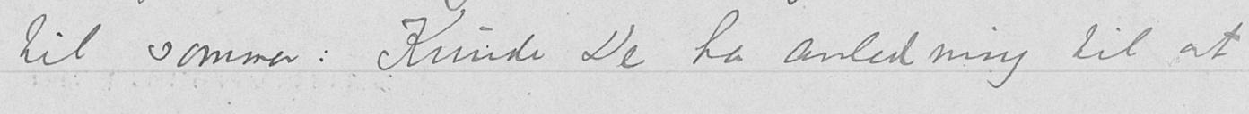til sommer: Kunde De ha anledning til at
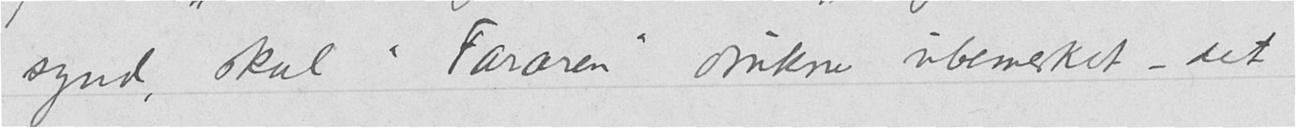synd, skal «Fararen" drukne ubemerket – det
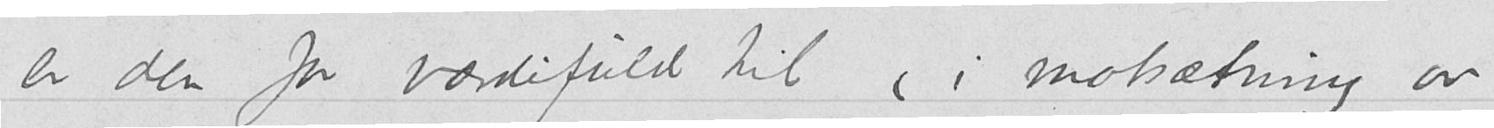er den for værdifuld til (i motsætning av
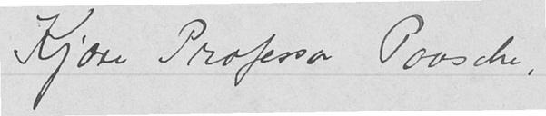Kjære Professor Paasche,
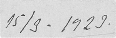15/3-1923.
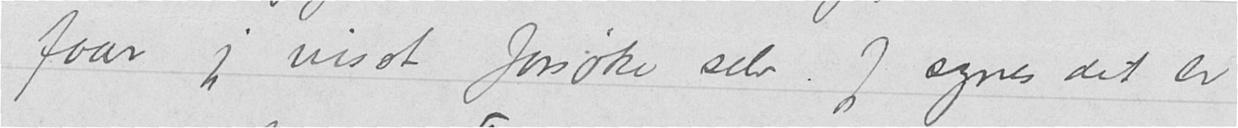faar jeg visst forsøke selv. Jeg synes det er
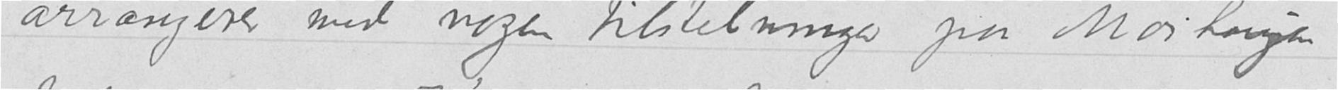arrangerer med nogen tilstelninger paa Maihaugen
